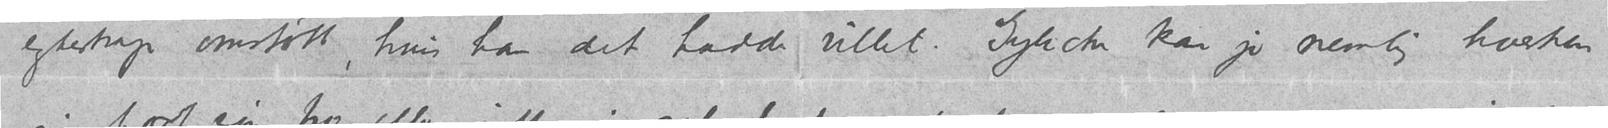egteskap omstøtt, hvis han det hadde villet. Gylicke kan jo nemlig hverken
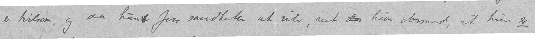er tvilsom, og da hun faar sandheten at vite, vet de hun dermed, at hun er
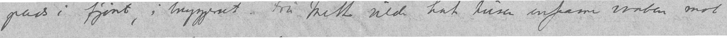plads i fjøset, i bryggerhuset.  Fru Mette vilde hat tusen infame vaaben mot
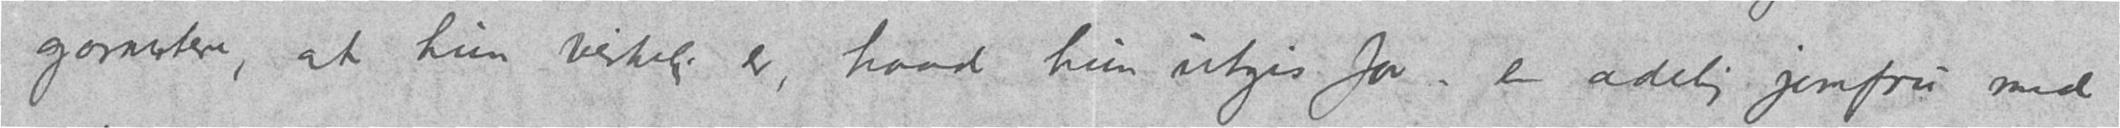garantere, at hun virkelig er, hvad hun utgis for – en adelig jomfru med
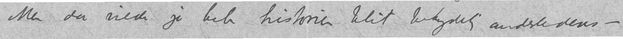Men da vilde jo hele historien blit betydelig anderledens – .
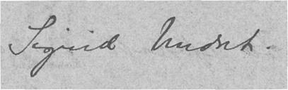Sigrid Undset.
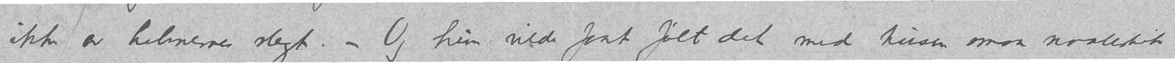ikke av Lehmernes slegt. – Og hun vilde faat følt det med tusen smaa naalestik
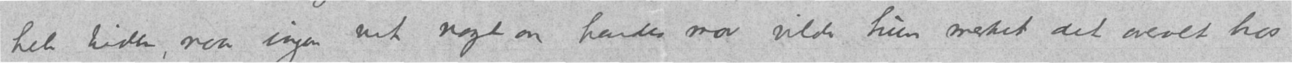hele tiden, naar ingen vet noget om hendes mor vilde hun merket det overalt hos
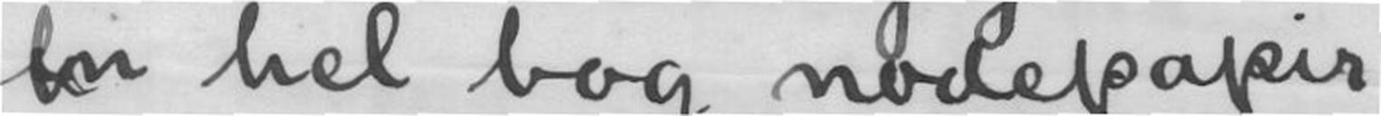en hel bog nodepapir
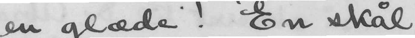en glæde! En skål,
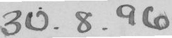30.8.96
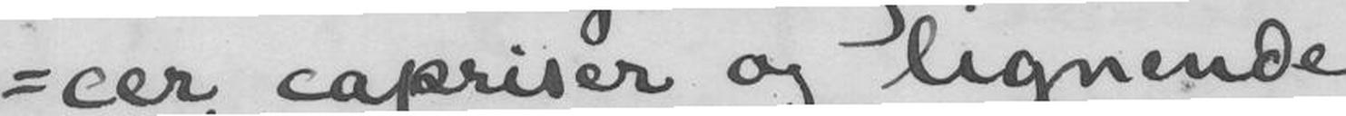cer, capriser og lignende
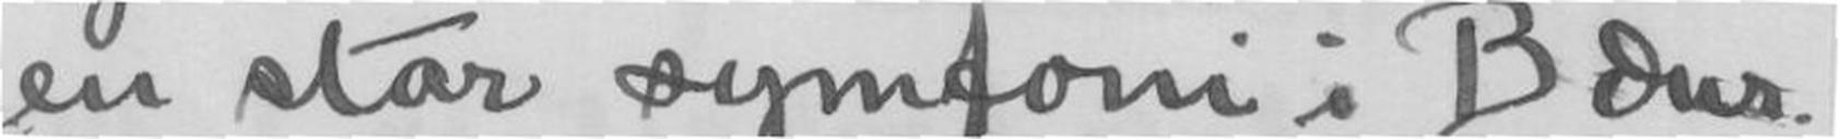en stor symfoni i B Dur.
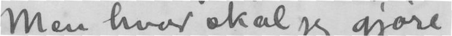Men hvad skal jeg gjøre
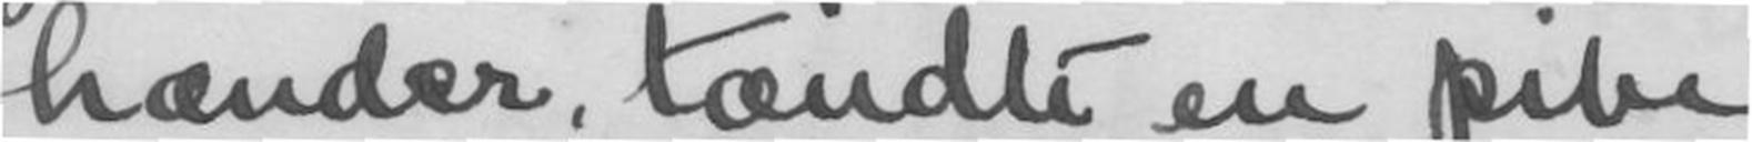hænder, tændte en pibe
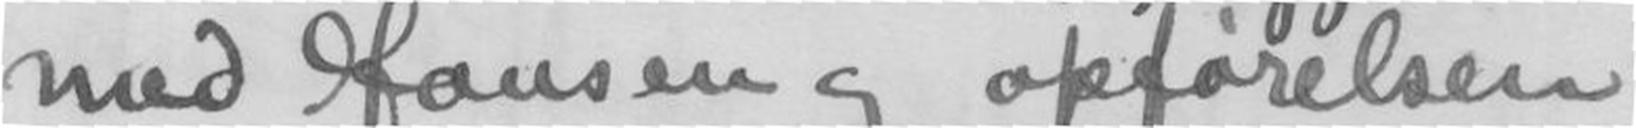med Hansen og opførelsen
